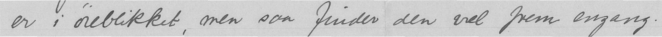er i øieblikket, men saa finder den vel frem engang.
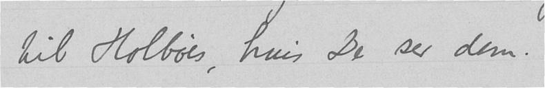til Holbøes, hvis De ser dem.
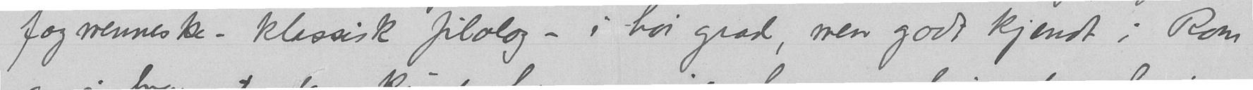fagmenneske - klassisk filolog - i høi grad, men godt kjendt i Rom
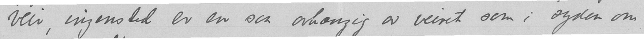veir, ingensted er en saa avhængig av veiret som i syden om
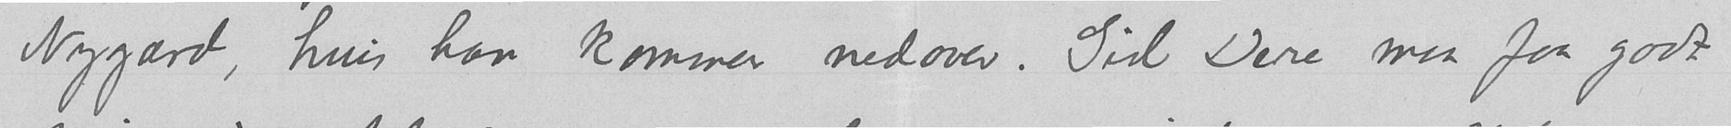Nygard, hvis han kommer nedover. Gid Dere maa faa godt
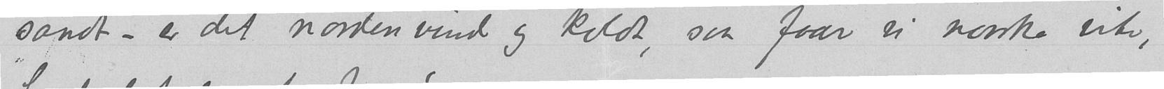sandt – er det nordenvind og koldt, saa faar vi norske vite,
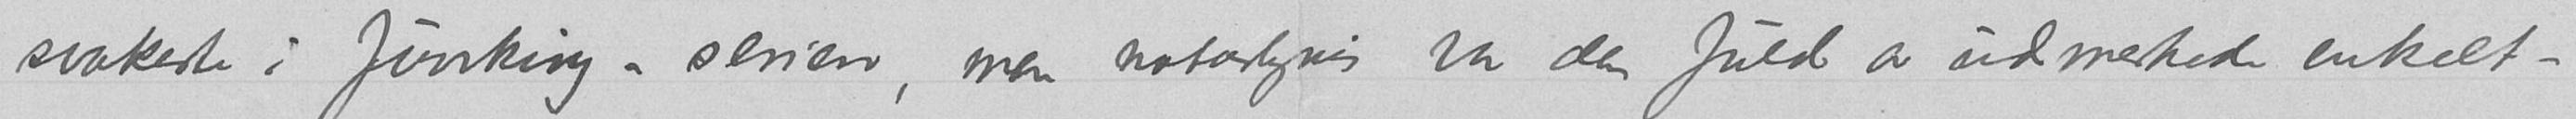svakeste i Juviking-serien, men naturligvis var den fuld av udmerkede enkelt-
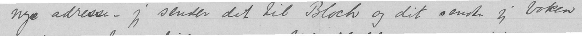nye adresse – jeg sender det til Bloch og dit sendte jeg boken
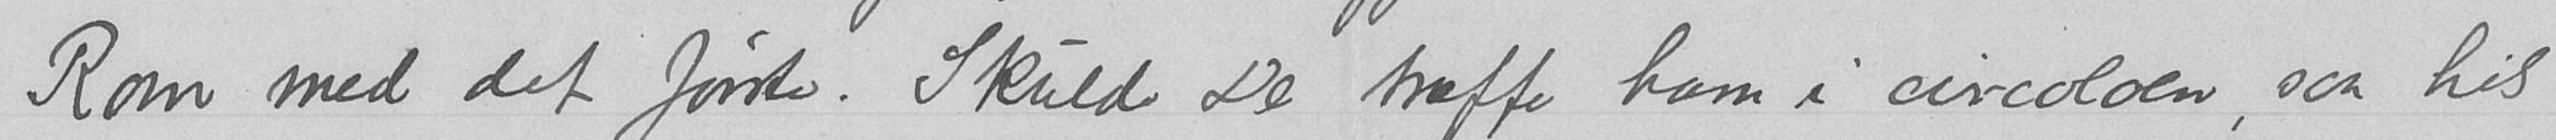Rom med det første. Skulde De træffe ham i circoloen, saa hils
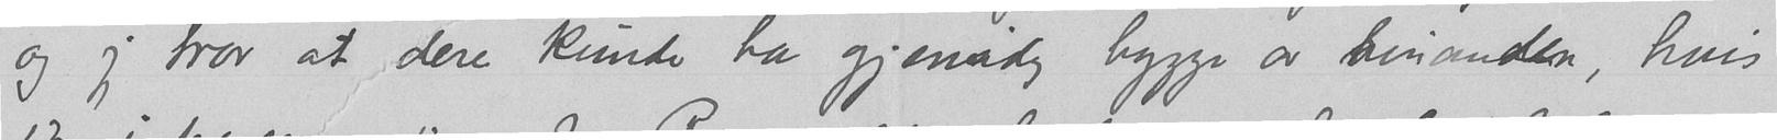og jeg tror at dere kunde ha gjensidig hygge av hinanden, hvis
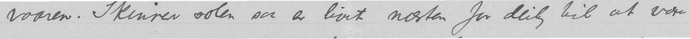vaaren. Skinner solen saa er livet næsten for deilig til at være
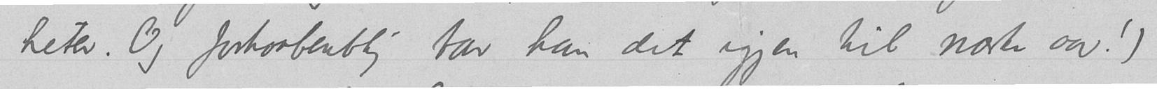heter. Og forhaabentlig tar han det igjen til næste aar!)
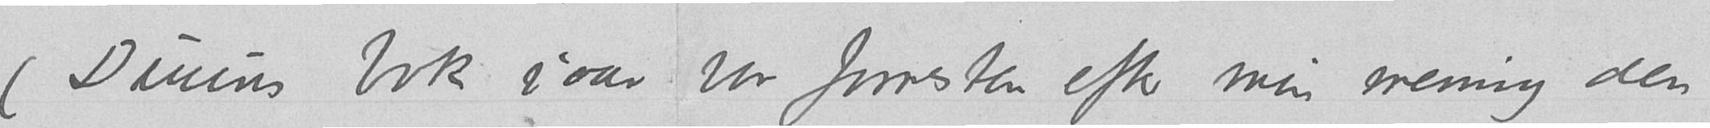(Duuns bok iaar var forresten efter min mening den
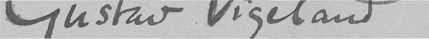Gustav Vigeland
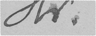Hr.
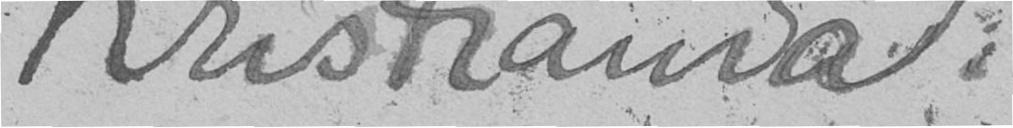Kristiania.
